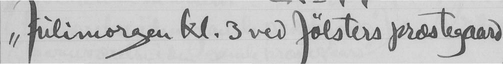"Julimorgen kl. 3 ved Jølsters præstegaard
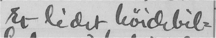Et lidet høidebil-
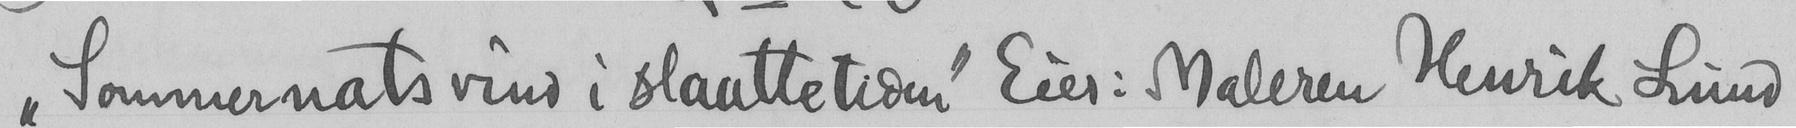"Sommernatsvind i slaattetiden" Eier: Maleren Henrik Lund
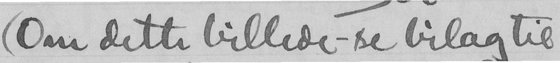(Om dette billede - se bilag til
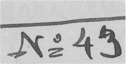No 43
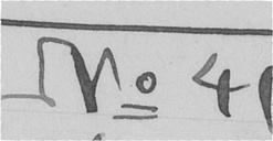No 41
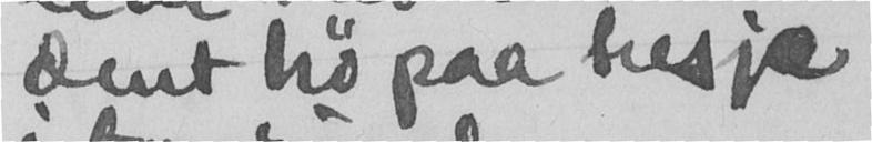denet hø paa hesje
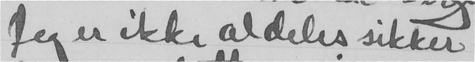Jeg er ikke aldeles sikker
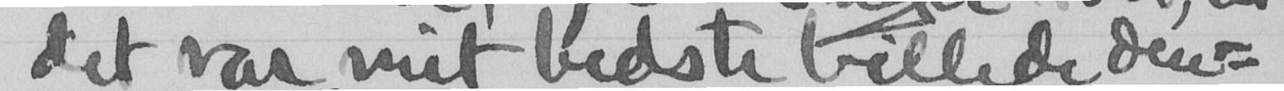det var mit bedste billede den-
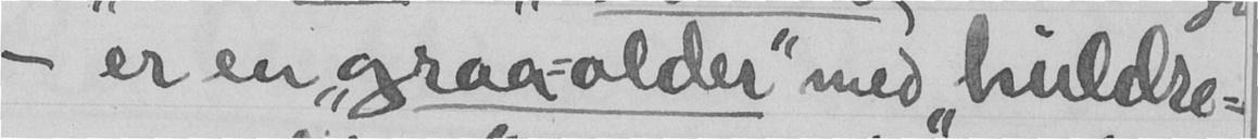- er en "graa-alder" med "huldre-
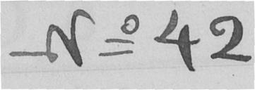No 42
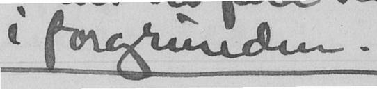i forgrunden.
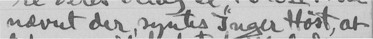nævnt der, syntes Inger Høst, at
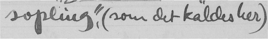sopling", (som det kaldes her)
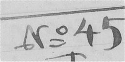No 45
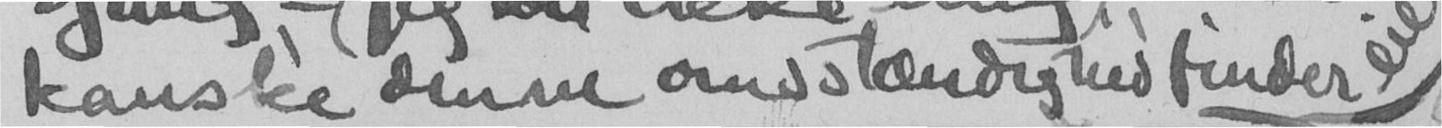kanske denne omsstændighed finder
